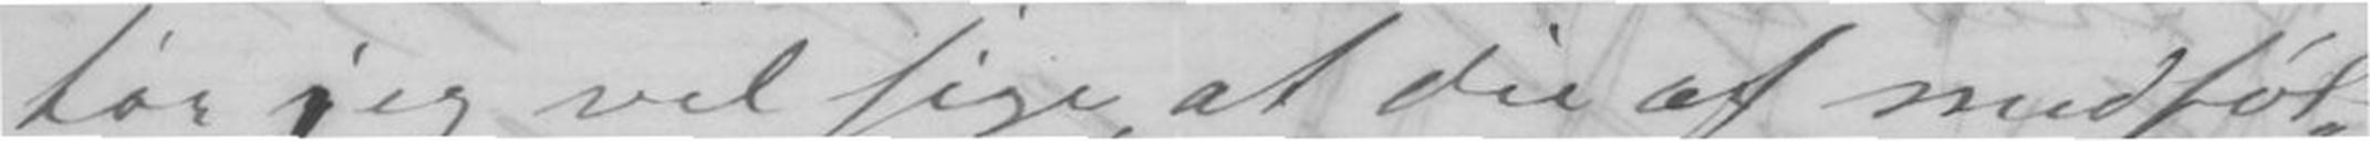tør jeg vel sige, at du af medføl-
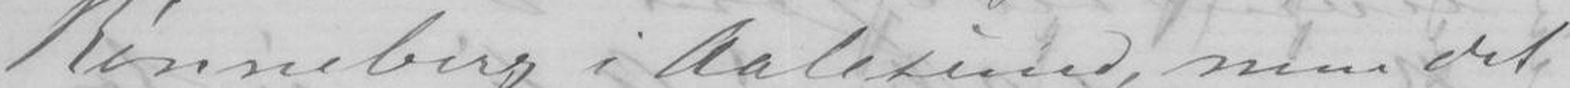Rønneberg i Aalesund, men det
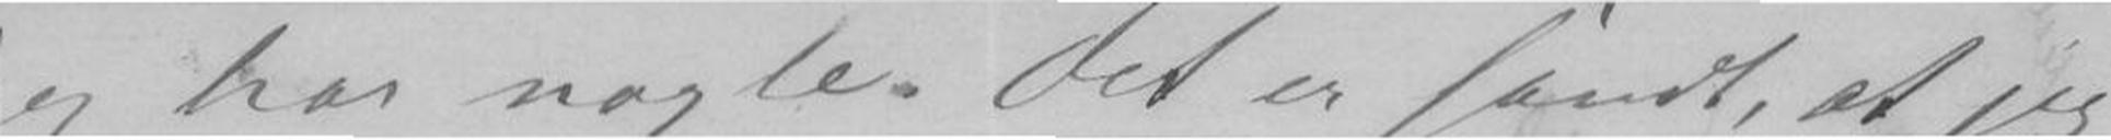jeg har nogle. Det er sandt, at jeg
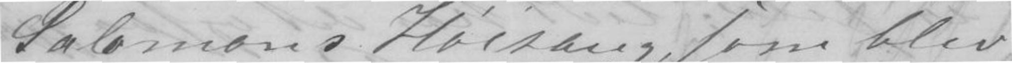Salomons Høisang, som blev
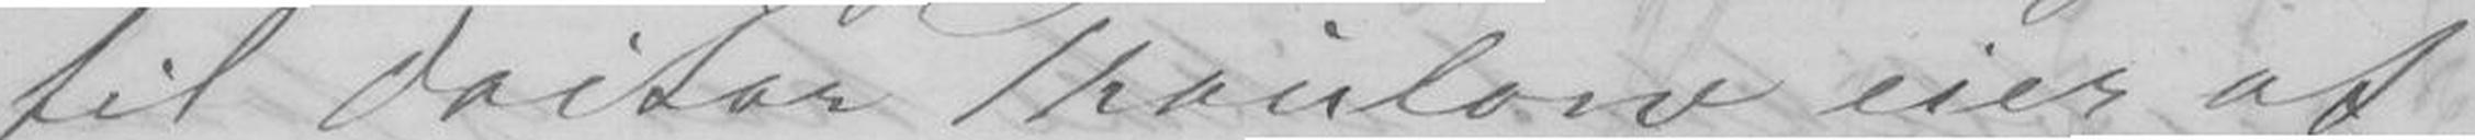til Doctor Thaulow eier af
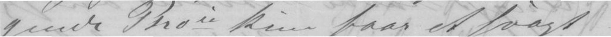gende Phoie kun faar et svagt
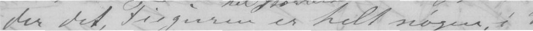der det, Figuren er helt nøgen, i
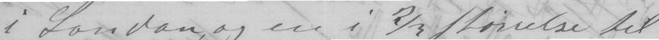i London, og en i 2/3 størrelse til
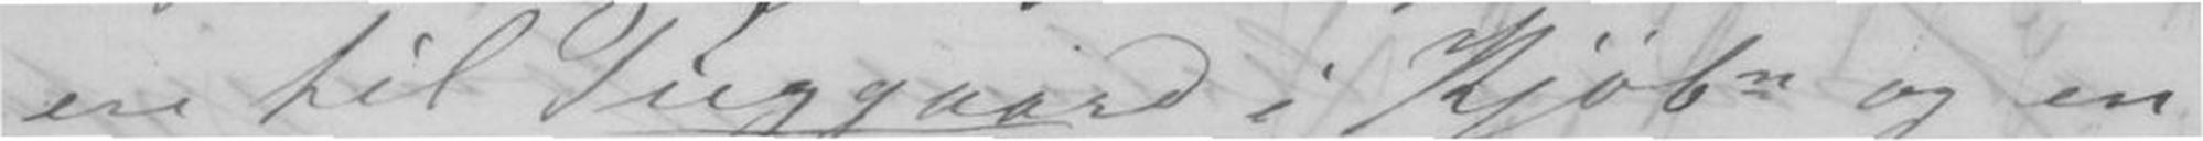en til Puggaard i Kjøbn og en
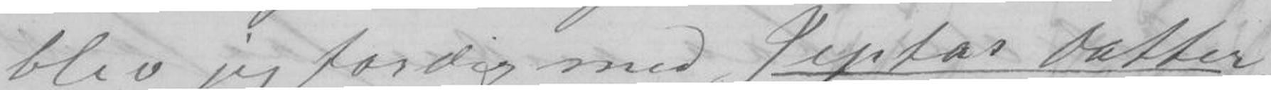blev jeg færdig med Jeftas Datter,
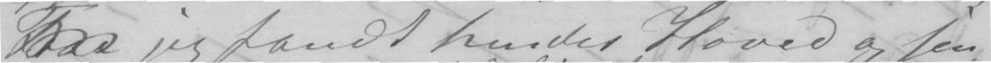jeg fandt hendes Hoved og sen-
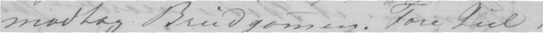modtag Brudgommen. Fore Jul
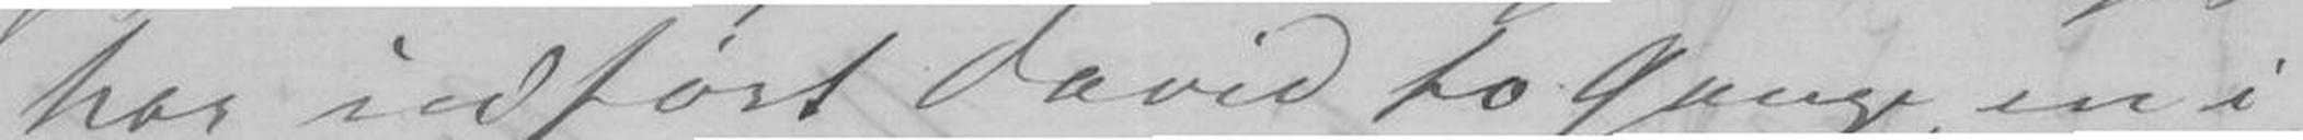har indført David to Gange, en i
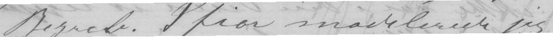Begreb. Ifior modelerede jeg
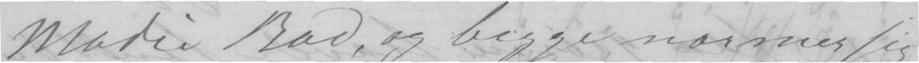Madie Bad, og begge nærmer sig
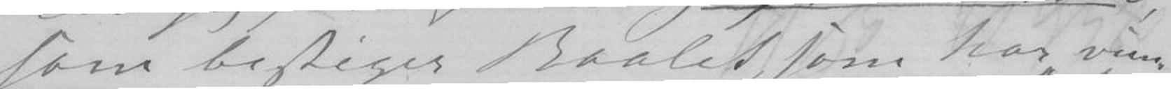som bestiger Baalet som har vun
